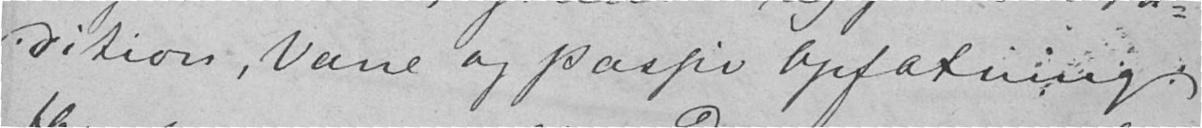dition, Vane og passiv Opfatning.
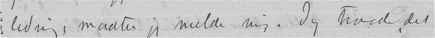ledning, maatte jeg melde mig. Jeg troede, det
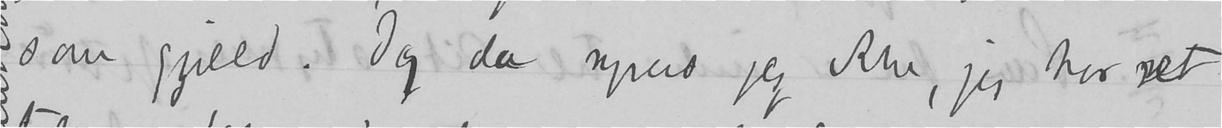som gjæld. Ja, da synes jeg ikke, jeg har ret
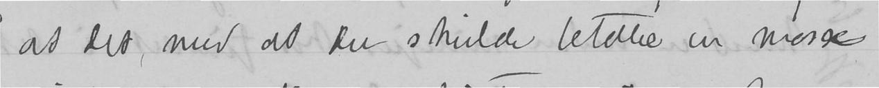at det, med at du skulde betale en masse
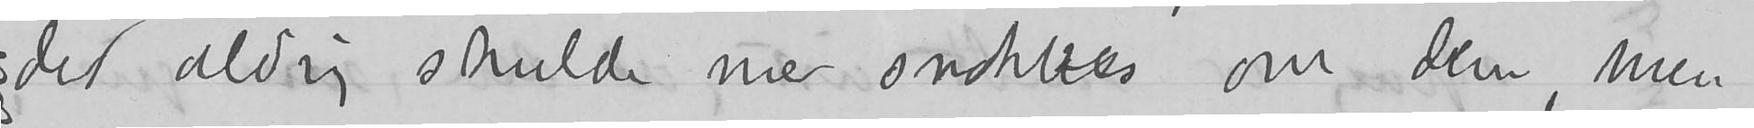det aldrig skulde mer snakkes om dem, men
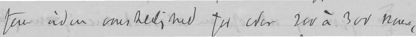fare uden vanskelighed for eder 200 à 300 kroner,
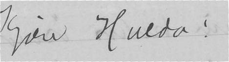Kjære Hulda!
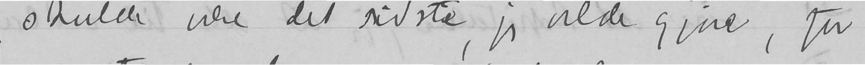skulde være det sidste, jeg vilde gjøre, for
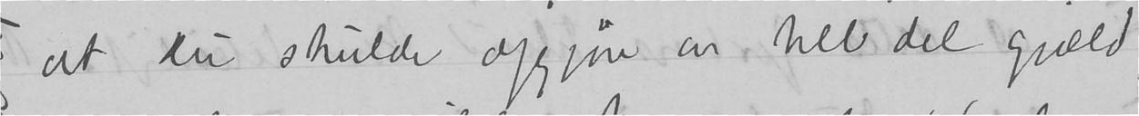at du skulde afgjøre en hel del gjæld,
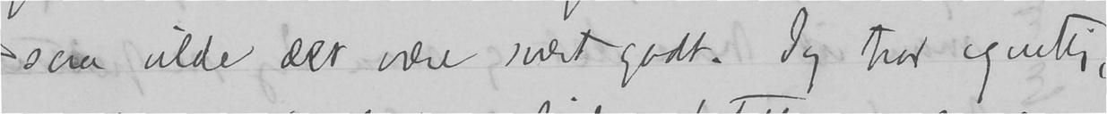saa vilde det være svært godt. Jeg tror egentlig,
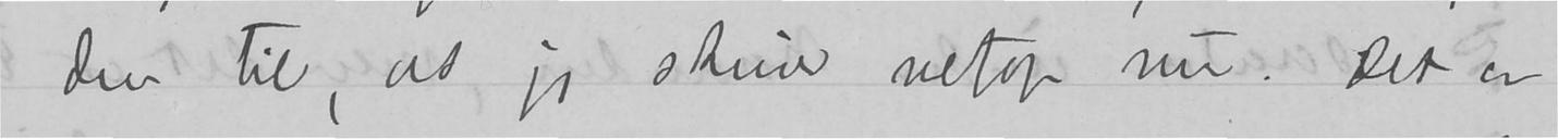den til, at jeg skriver netop nu. Det er
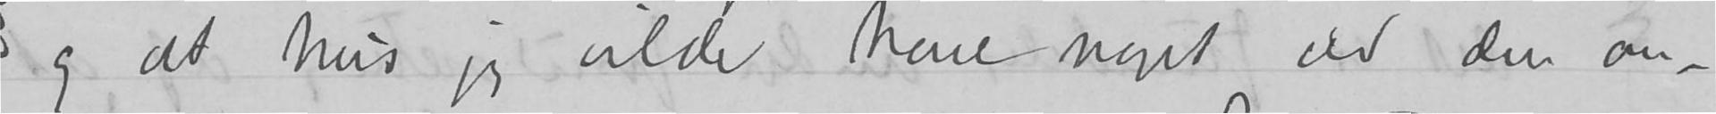og at hvis jeg vilde have noget ved den an-
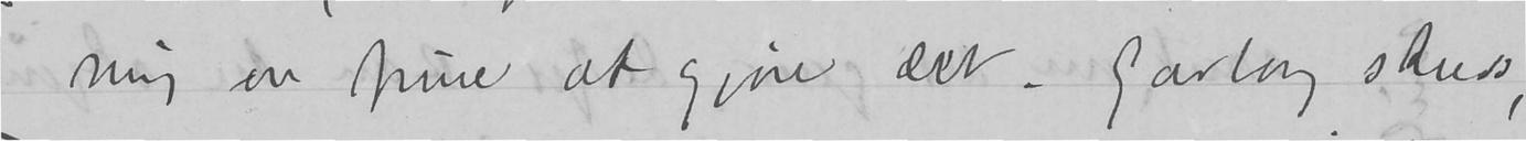mig en pine at gjøre det. Garborg skrev,
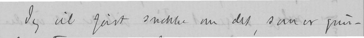Jeg vil først snakke om det, som er grun-
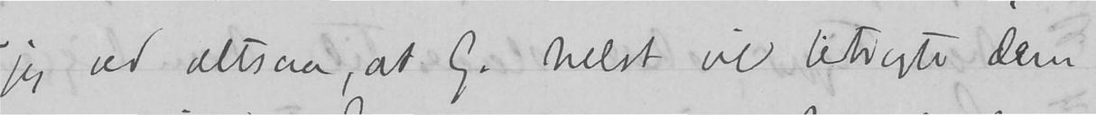jeg ved altsaa, at G. helst vil betragte dem
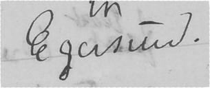Egersund.
